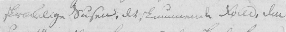skrækelige Susen, det skummende Fald, den
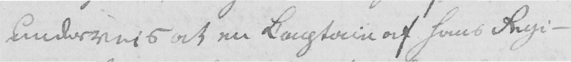underveis at en Captain af hans Regi-
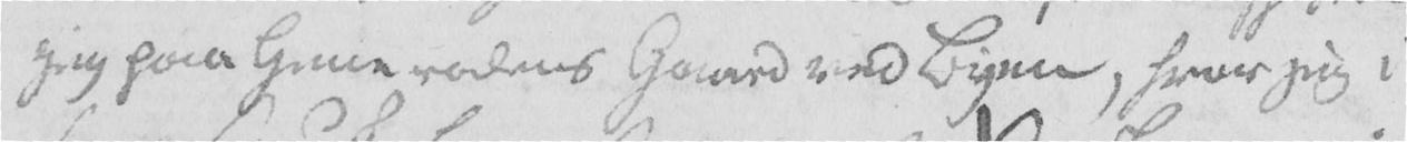jeg paa Generalens Gaard ved Byen, hvor jeg i
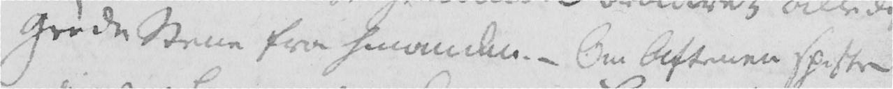Grøde Stene fra hinanden. - Om Aftenen spiste
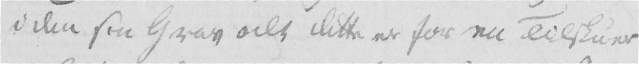i den sin Grav alt dette er for en Tilskuer
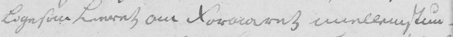Ligesom Leeret om Foraaret mellemstun-
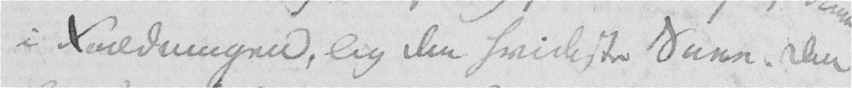i Faldningen, lig den hvideste Snee. Den
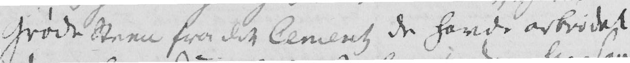Grøde Steen fra det Cement de havde arbeidet
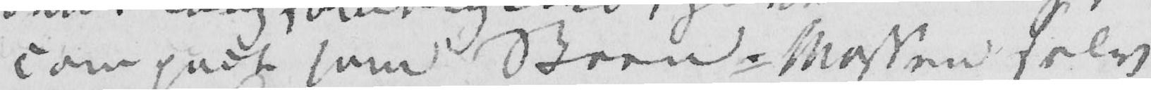compact som Steen-Massen selv
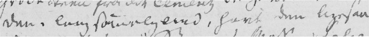den i langsomelig Tiid, havt den ligesaa
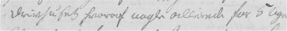Drivhuset hvoraf nogle allerede for 5 Uger
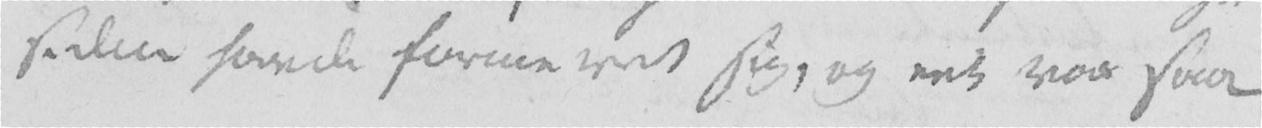siden havde formeret sig, og eet var saa
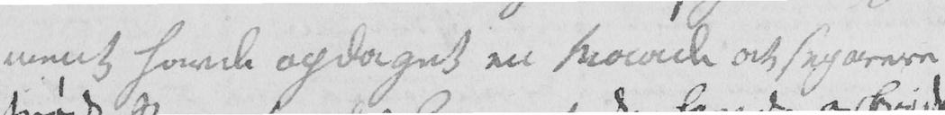ment havde opdaget en Maade at separere
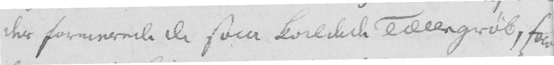der formerede de saa kaldede Tællegrøb, for
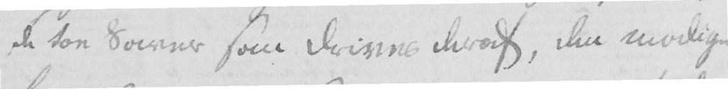de toe Saver som drives deraf, den modige
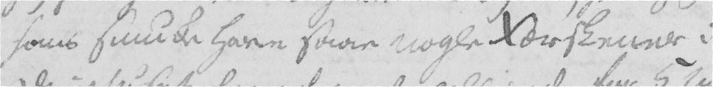hans smucke Have saae nogle Færskener i
